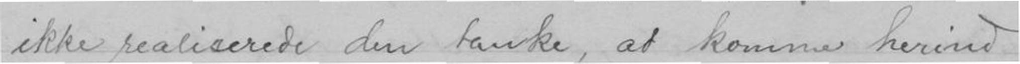ikke realiserede den tanke, at komme herind
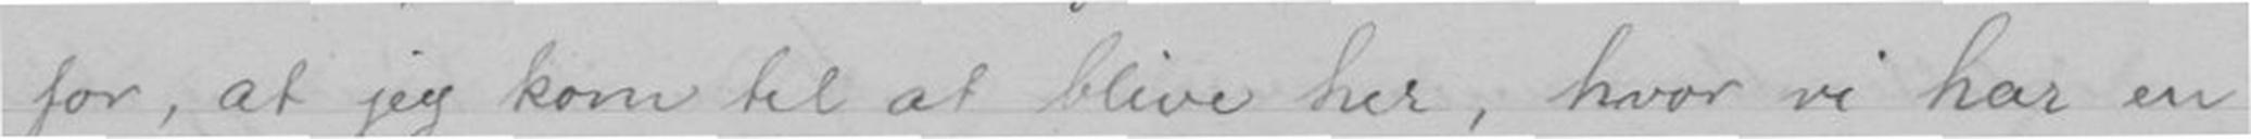for, at jeg kom til at blive her, hvor vi nu har en
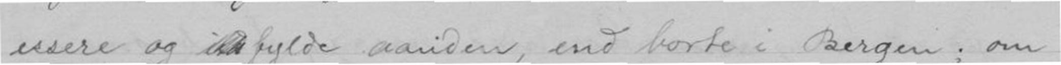ressere og fylde aandten, end borte i Bergen; om
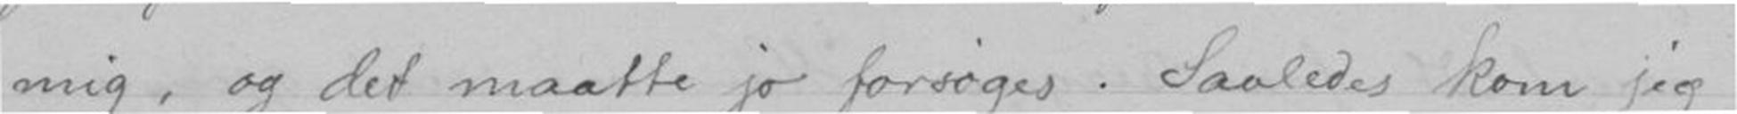mig, og det maatte jo forsøges. Saaledes kom jeg
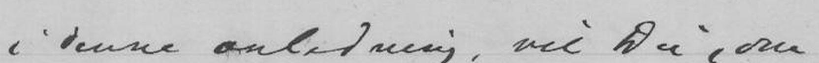i denne anledning, vil Du, om Du
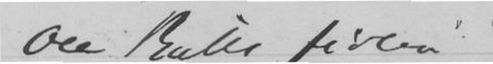Ole Bulls fiolin
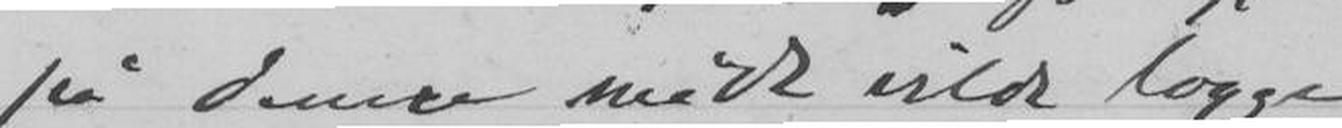på denne måde vilde lægge
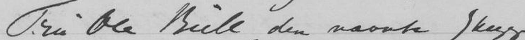Fru Ole Bull "den nævnte Skugg-
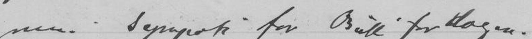min sympati for Bull for Dagen.
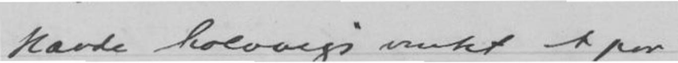Havde halvvejs ventet et par
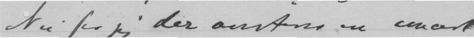Nu ser jeg der averteres en concert
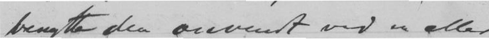benytte den anvendt ved en eller
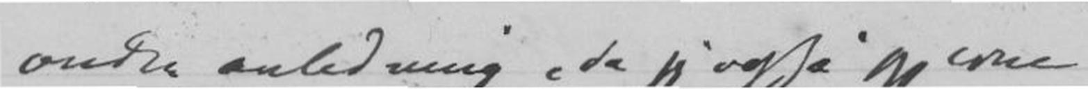anden anledning da jeg også gjerne
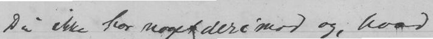ikke har noget imod og, hvad
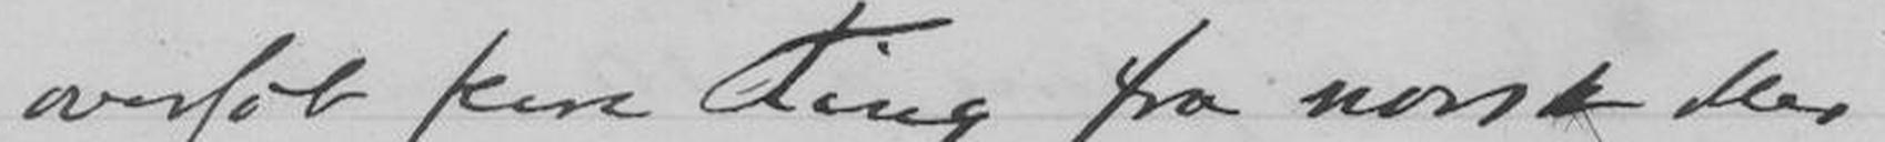oversat flere Ting fra norsk eller
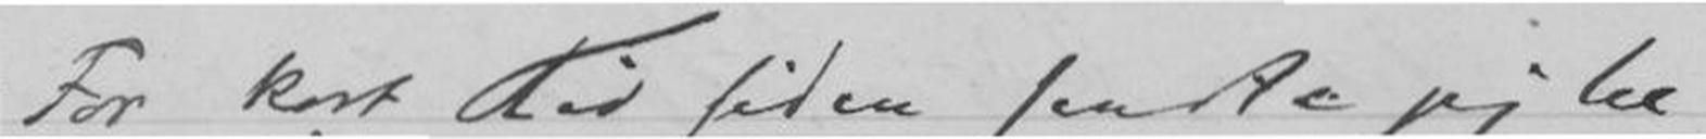For kort Tid siden sendte jeg til
